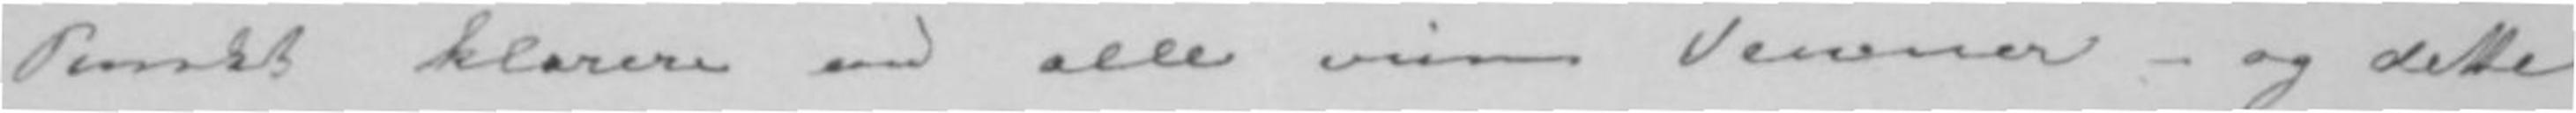Punkt klarere end alle mine Venner- og dette
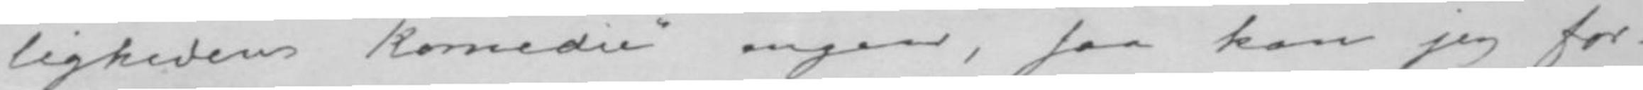lighedens Komedie» angaar, saa kan jeg for-
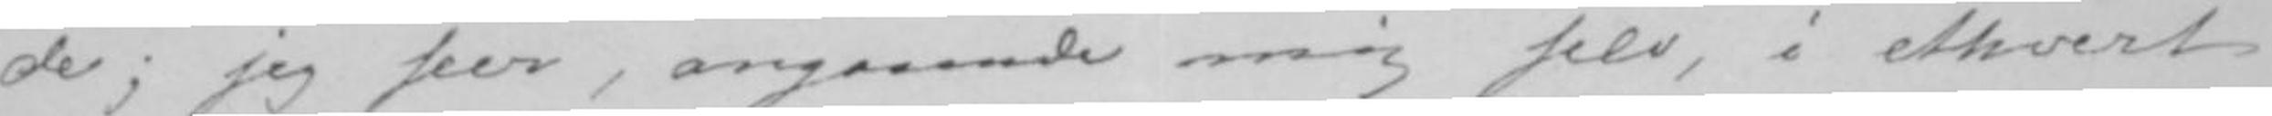de; jeg seer, angaaende mig selv, i ethvert
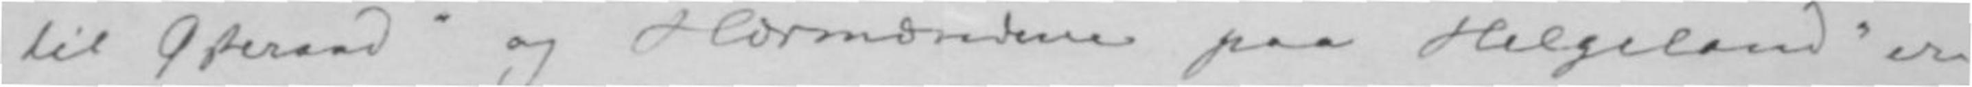til Østeraad» og «Hærmændene paa Helgeland» er
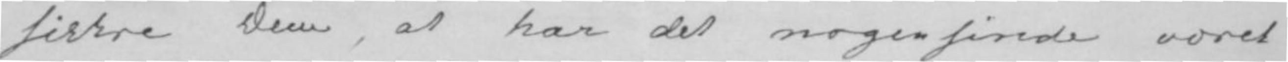sikkre Dem, at har det nogensinde været
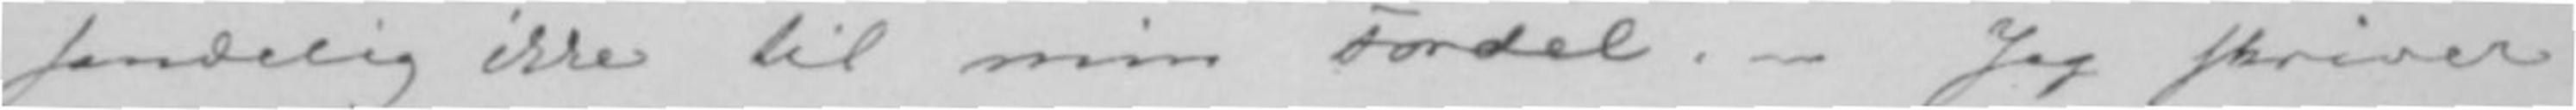sandelig ikke til min Fordel.- Jeg skriver
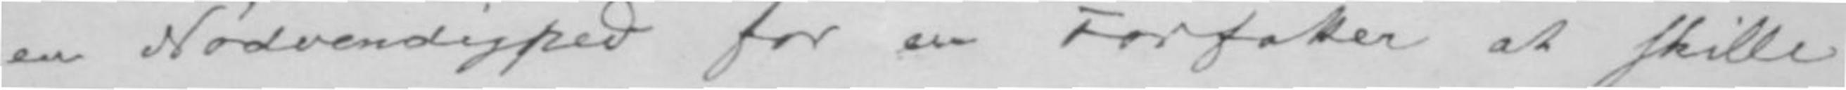en Nødvendighed for en Forfatter at skille
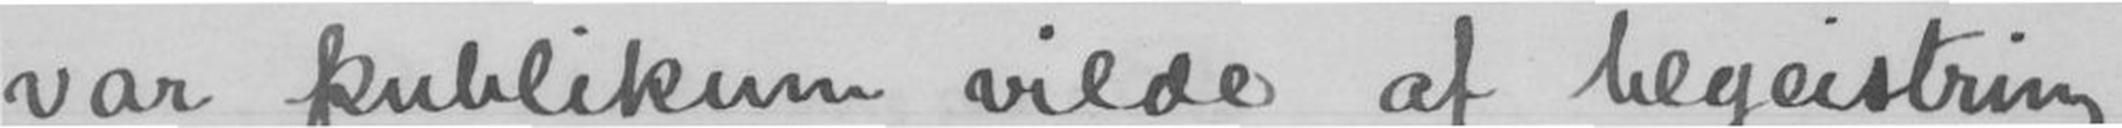var publikum vilde af begeistring
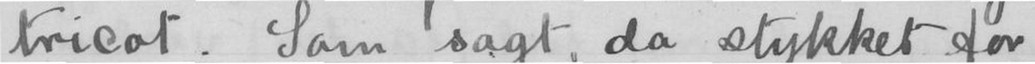tricot. Som sagt, da stykket for
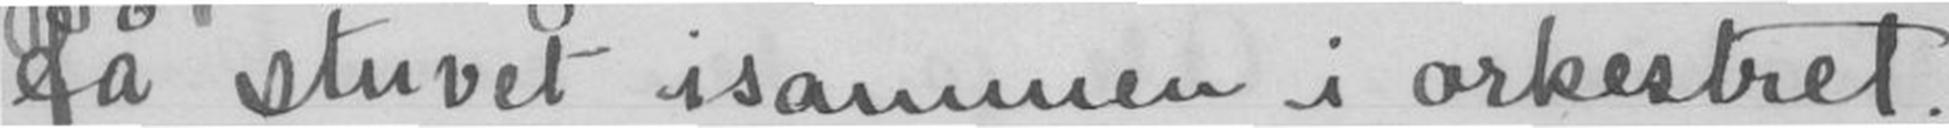få stuvet isammen i orkesteret.
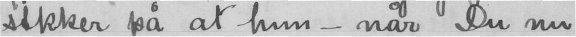sikker på at hun - når Du nu
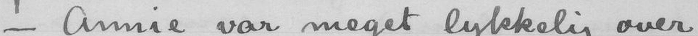- Annie var meget lykkelig over
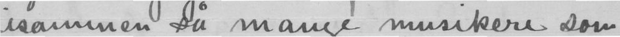isammen så mange musikere som
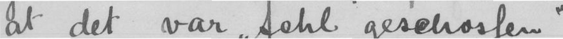at det var fehl geschossen.
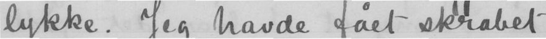lykke. Jeg havde fået skrabet
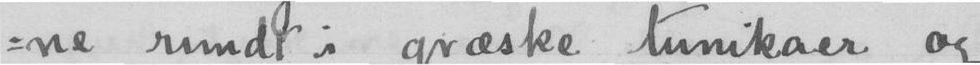=ne rundt i græske tunikaer og
